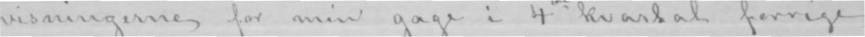visningerne for min gage i 4de kvartal forrige
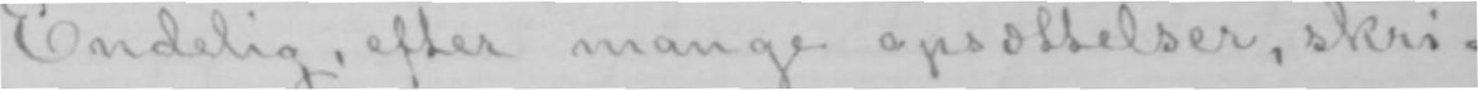Endelig, efter mange opsættelser, skri-
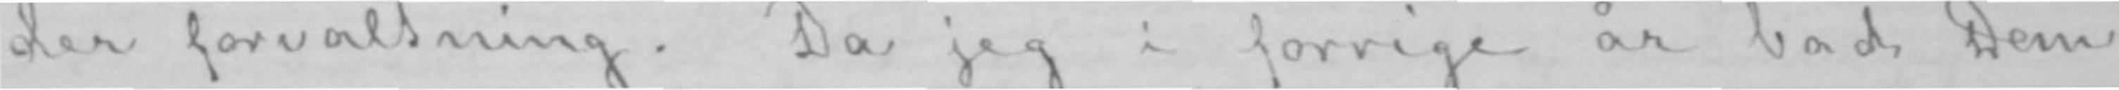der forvaltning. Da jeg i forrige år bad Dem
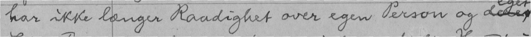har ikke længer Raadighet over egen Person og deres
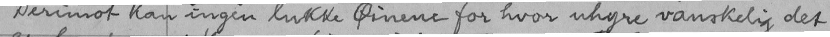Derimot kan ingen lukke Øinene for hvor uhyre vanskelig det
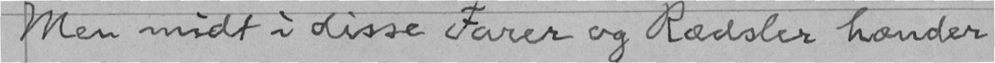Men midt i disse Farer og Rædsler hænder
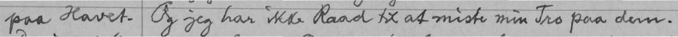paa Havet. Og jeg har ikke Raad til at miste min Tro paa dem.
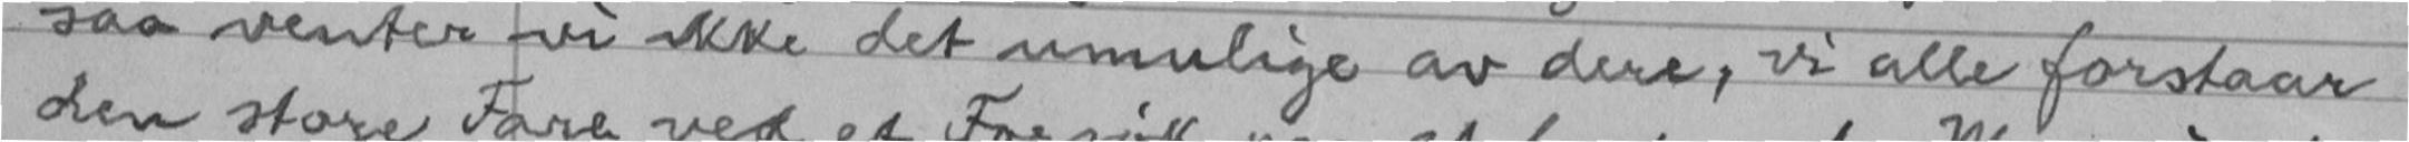saa venter vi ikke det umulige av dere, vi alle forstaar
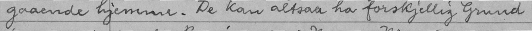gaaende hjemme. De kan altsaa ha forskjellig Grund
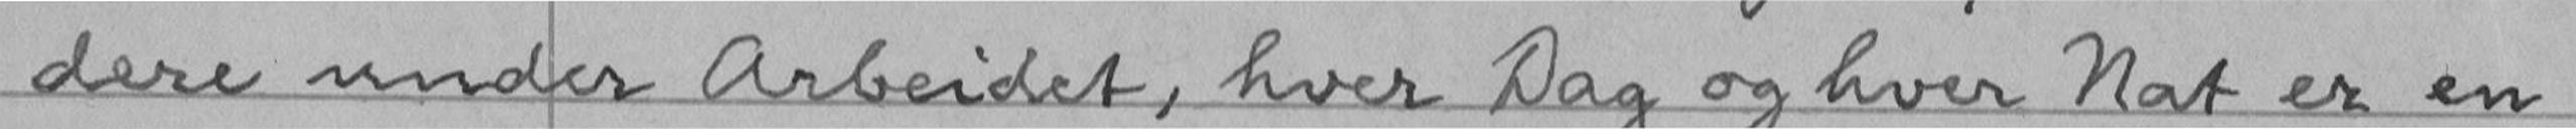dere under Arbeidet, hver Dag og hver Nat er en
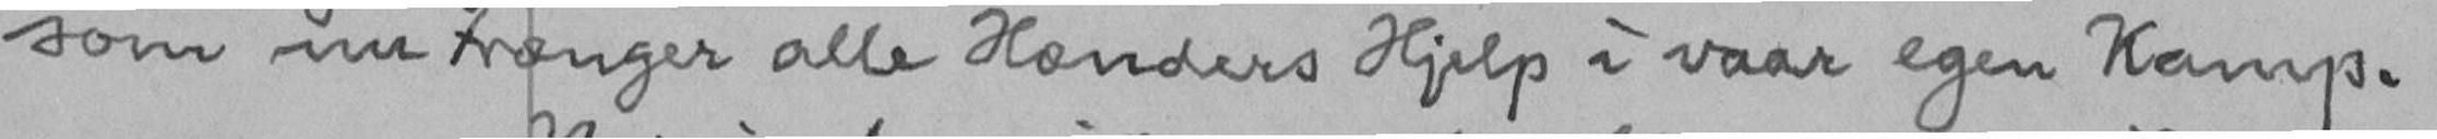som nu trænger alle Hænders Hjelp i vaar egen Kamp.
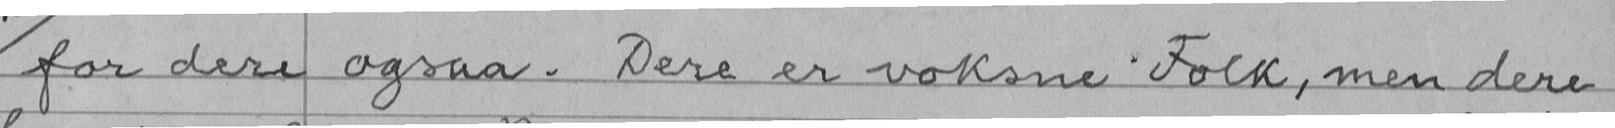for dere ogsaa. Dere er voksne Folk, men dere
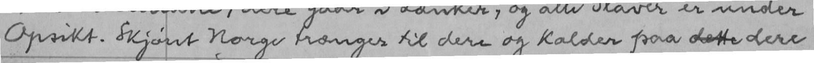Opsikt. Skjønt Norge trænger til dere og kalder paa dette dere
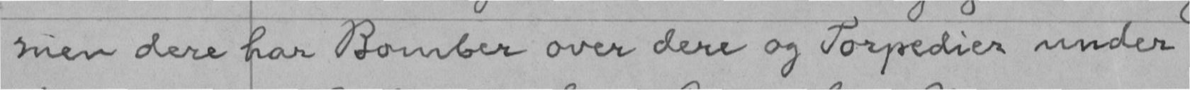men dere har Bomber over dere og Torpedier under
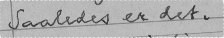Saaledes er det.
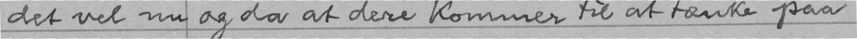det vel nu og da at dere kommer til at tænke paa
